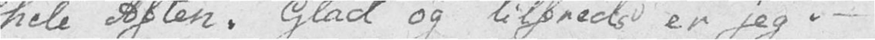hele Aften. Glad og tilfreds er jeg. –
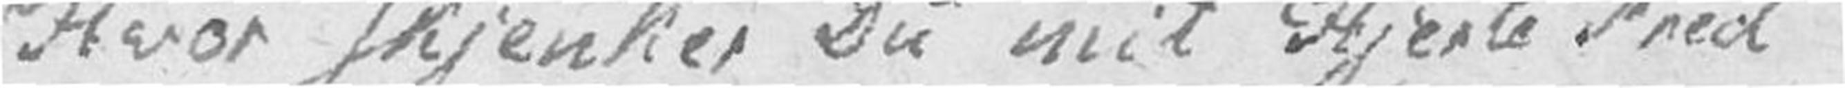Hvor skjenkes Du mit Hjerte Fred
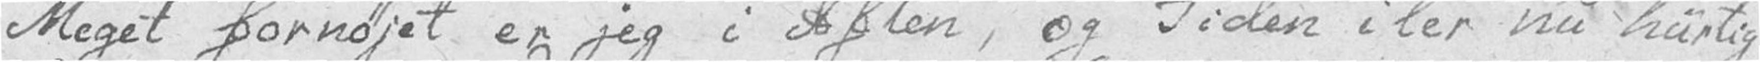Meget fornøjet er jeg i Aften, og Tiden iler nu hurtig
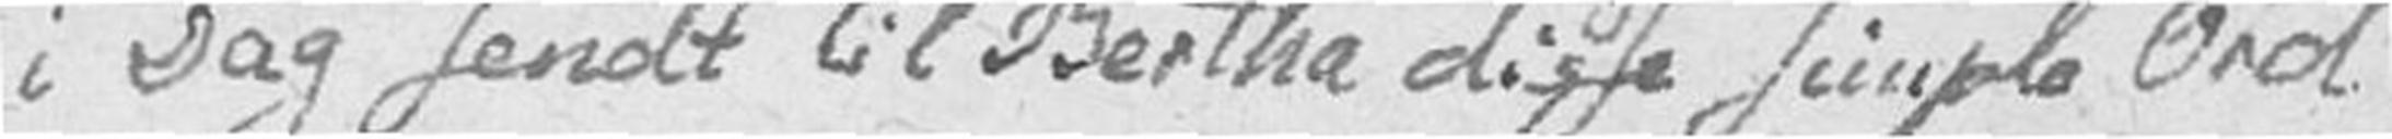i Dag sendt til Bertha disse simple Ord.
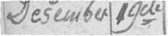Desember 19de
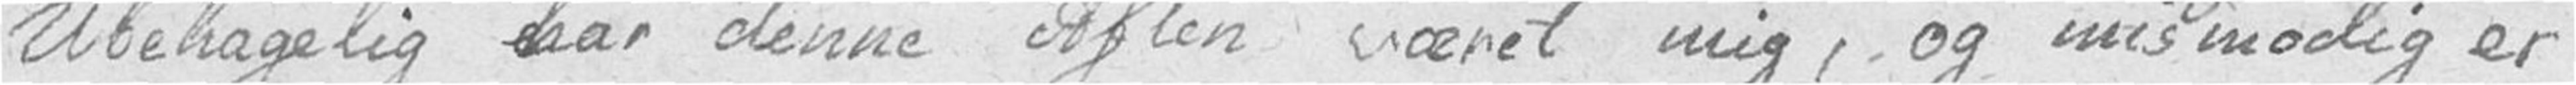Ubehagelig har denne Aften været mig, og mismodig er
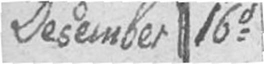Desember 16d
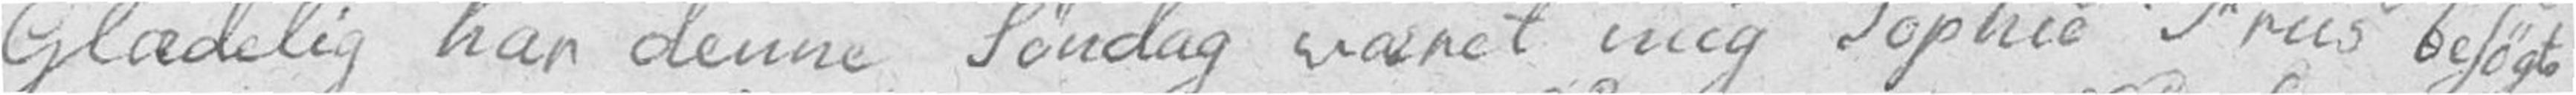Glædelig har denne Søndag været mig Sophie Friis besøgte
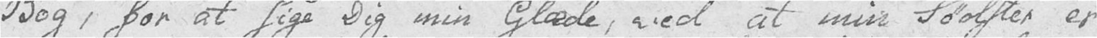Bog, for at sige Dig min Glæde, ved at min Sødster er
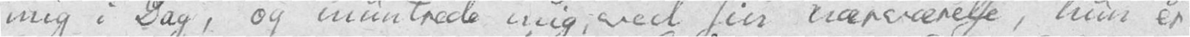mig i Dag, og muntrede mig, ved sin nærværelse, hun er
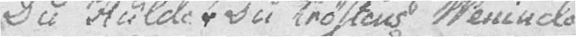Du Hulde! Du trøstens Veninde
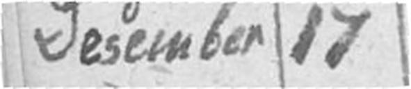Desember 17
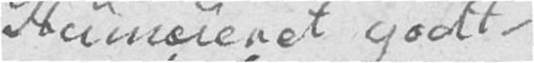Humeuret godt.
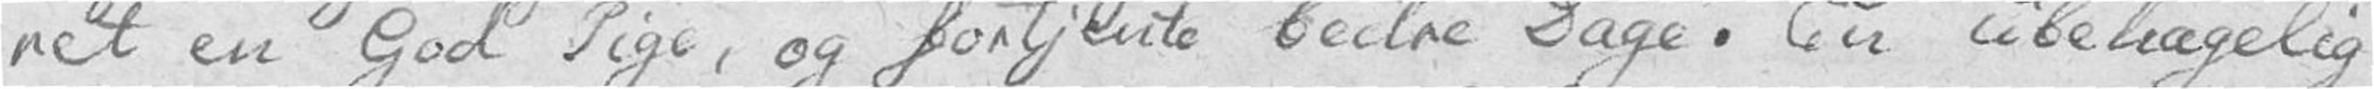ret en God Pige, og fortjente bedre Dage. En ubehagelig
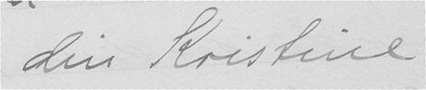din Kristine
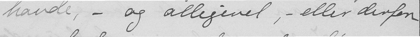havde, - og alligevel,- eller derfor
